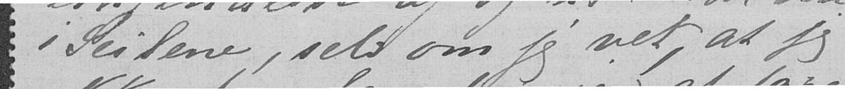i Seilene, selv om jeg vet, at jeg
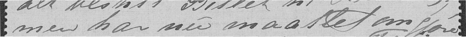men har nu maattet omgjøre
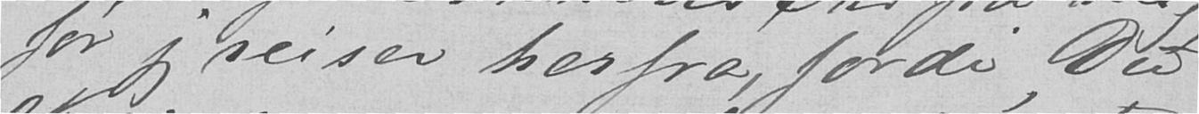før jeg reiser herfra, fordi Du
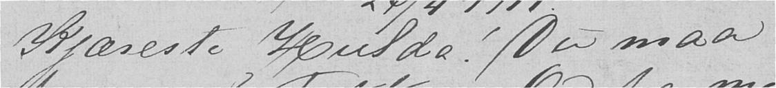Kjæreste Hulda! Du maa
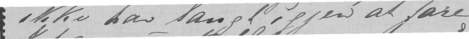ikke har langt igjen at fare.
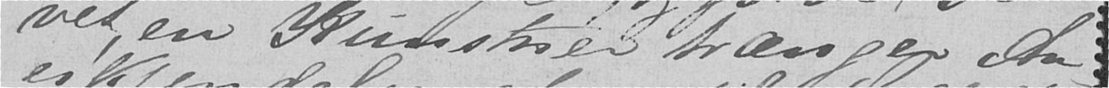vet, en Kunstner trænger An-
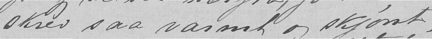skrev saa varmt og skjønt
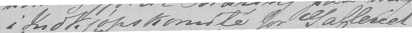i Indkjøbskomite for Galleriet
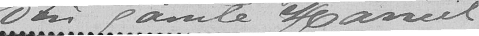Din gamle Harriet
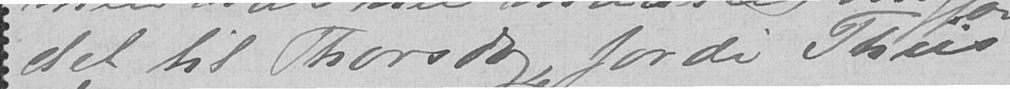det til Thorsdag, fordi Thiis
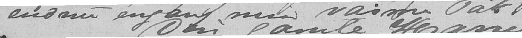endnu engang min varme Tak!
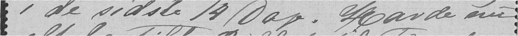i de sidste 14 Dage. Havde nu
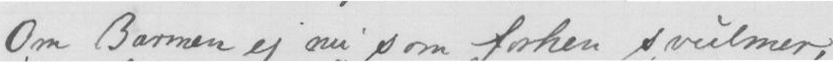Om Barmen ej nu som forhen svulmer,
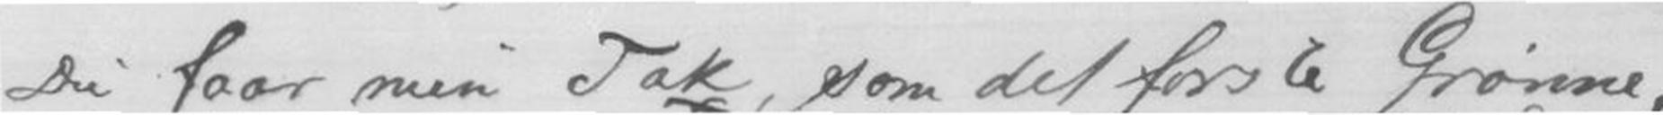Du faar min Tak, som det første Grønne,
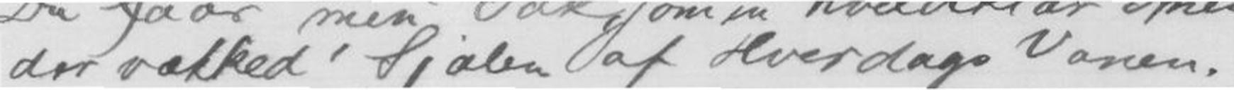der vætked' Sjælen af Hverdags Vanen.
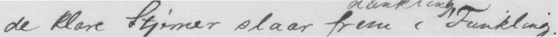de klare Stjerner slaar frem i Funkling.
